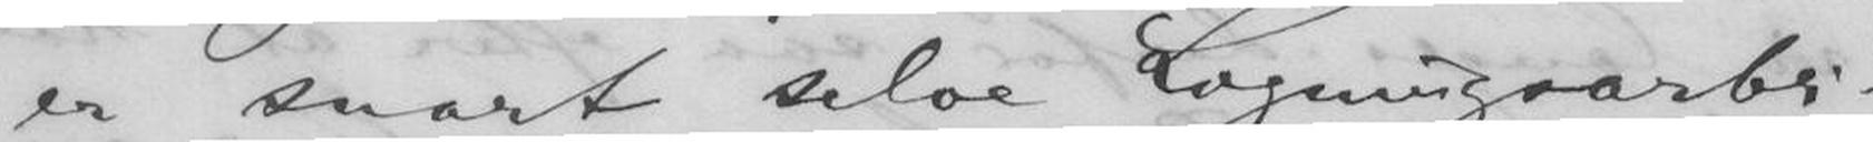er snart selve Ligningsarbei-
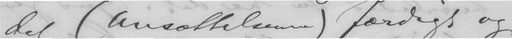det (Ansættelsen) færdigt og
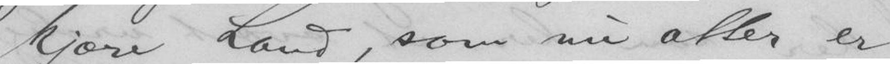kjære Land, som nu atter er
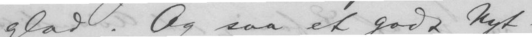glad. Og saa et godt Nyt-
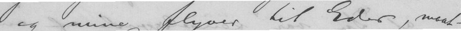og mine flyver til Eder, maat-
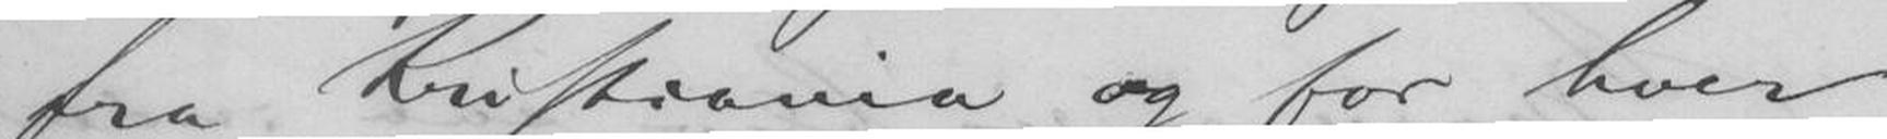fra Kristiania og for hver
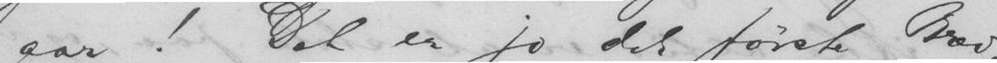aar! Det er jo det første Brev,
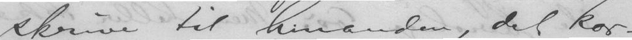skrive til hinanden, det kor-
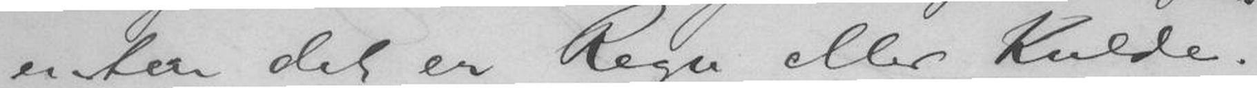enten det er Regn eller Kulde.
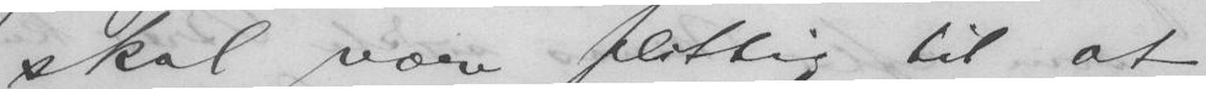skal være flittig til at
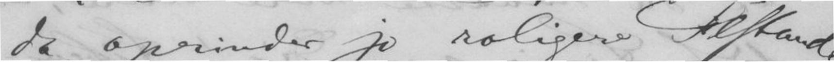da oprinder jo roligere Tilstande.
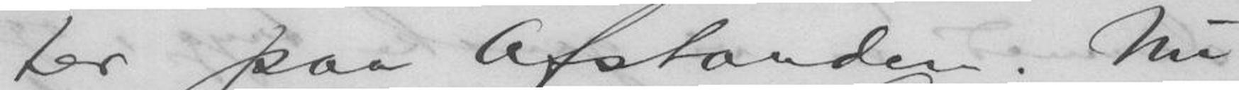ter paa Afstanden. Nu
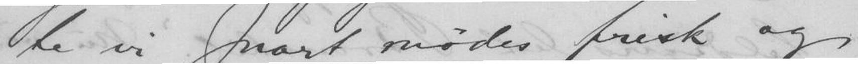te vi snart mødes frisk og
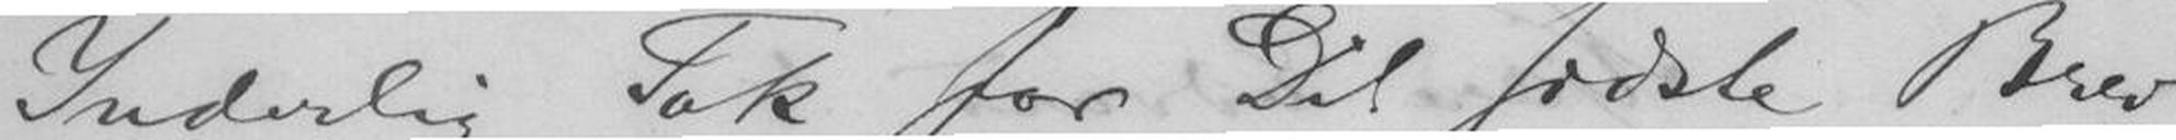Inderlig Tak for Dit sidste Brev
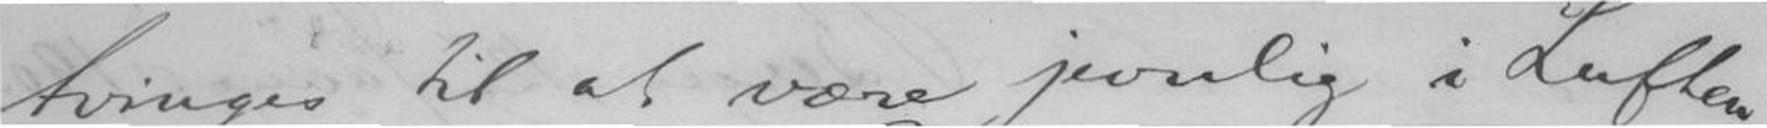tvinges til at være jevnlig i Luften
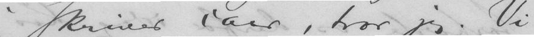jeg skriver iaar, tror jeg. Vi
